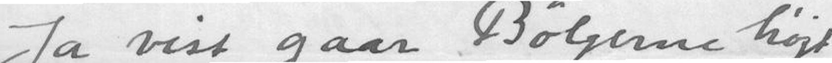Ja vist gaar Bølgerne højt
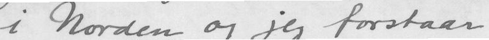i Norden og jeg forstaar
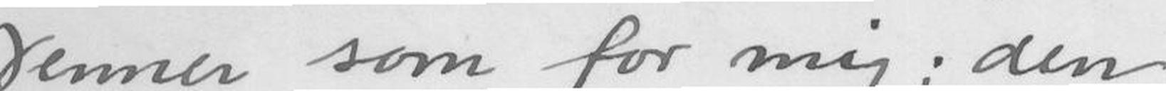Venner som for mig; den
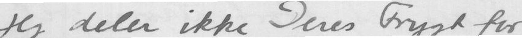jeg deler ikke Deres Frygt for
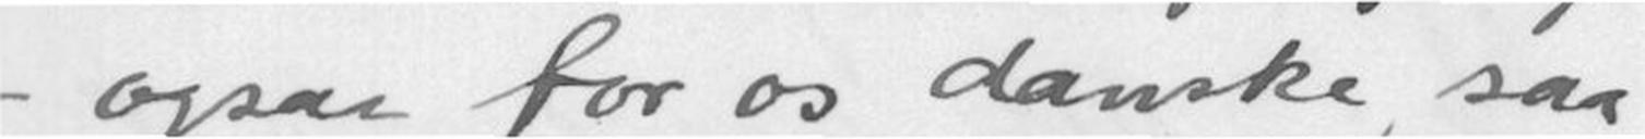ogsaa for os danske, saa
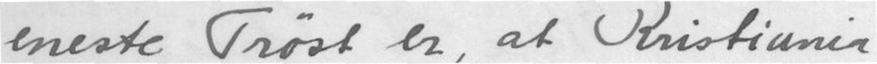eneste Trøst er, at Kristiania,
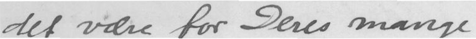det være for Deres mange
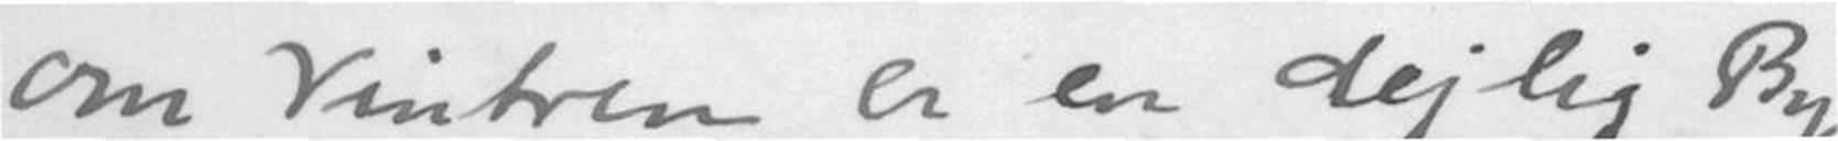om Vintren er en dejlig By
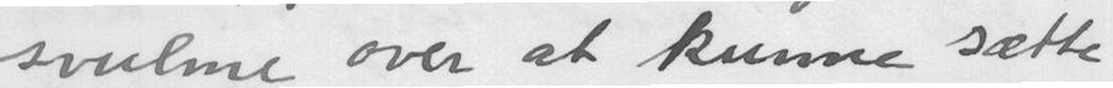svulme over at kunne sætte
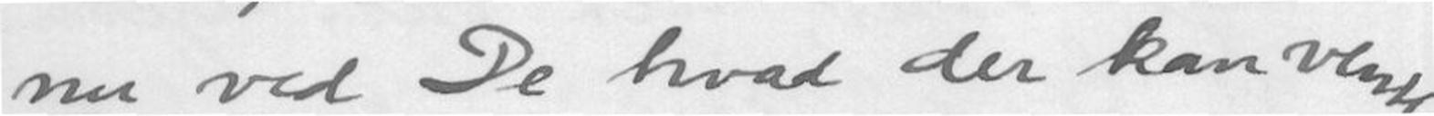nu ved De hvad der kan vente
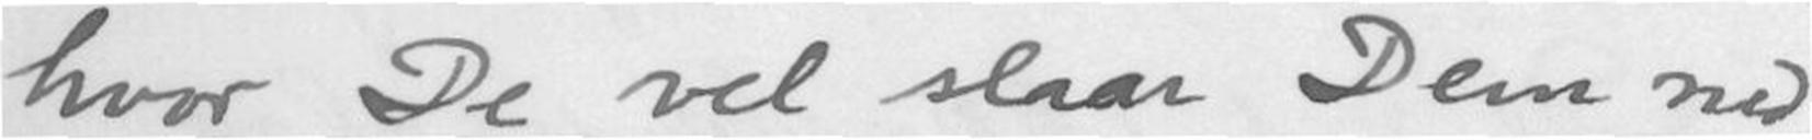hvor De vel slaar Dem ned
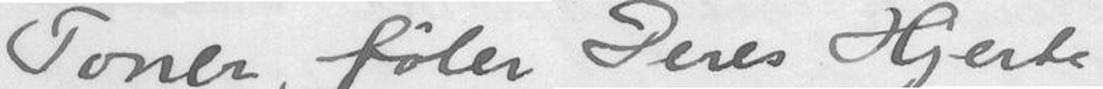Toner, føler Deres Hjerte
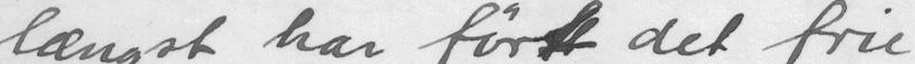længst har ført det frie
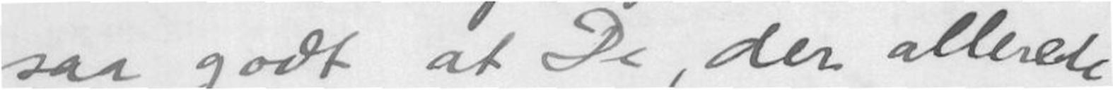saa godt at De, der allerede
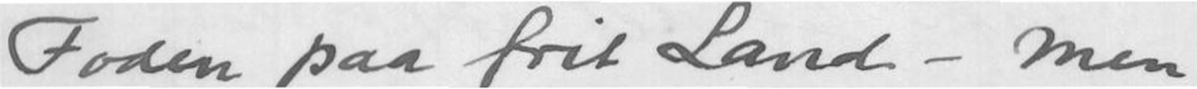Foden paa frit Land - men
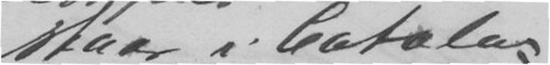staar i Catalo-
